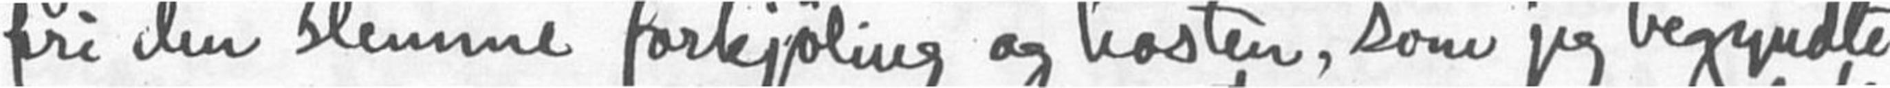fri den slemme forkjøling og hosten, som jeg begynte
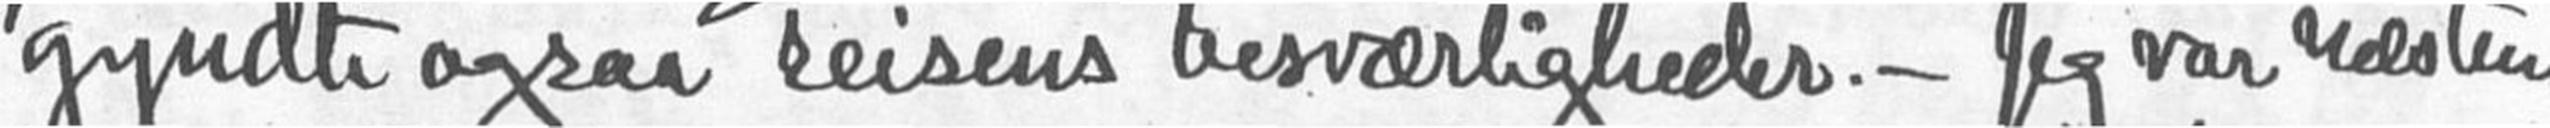gyndte ogsaa reisens besværligheder. - Jeg var næsten
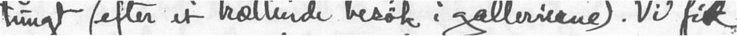tungt (efter et trættende besøk i gallerierne). Vi fik
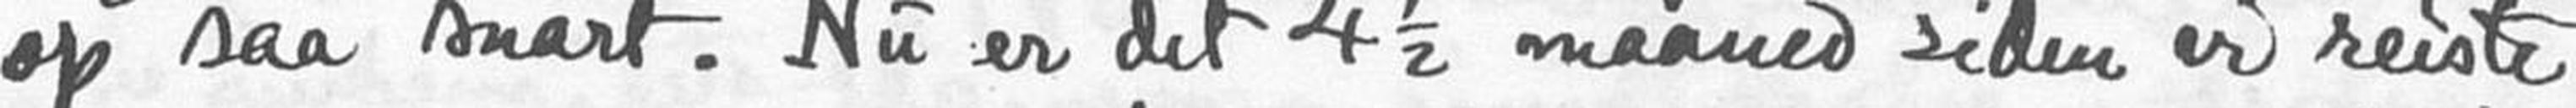op saa snart. Nu er det 4 1/2 maaned siden vi reiste
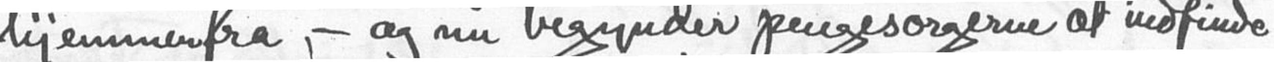hjemmenfra, - og nu begynder pengesorgerne at indfinde
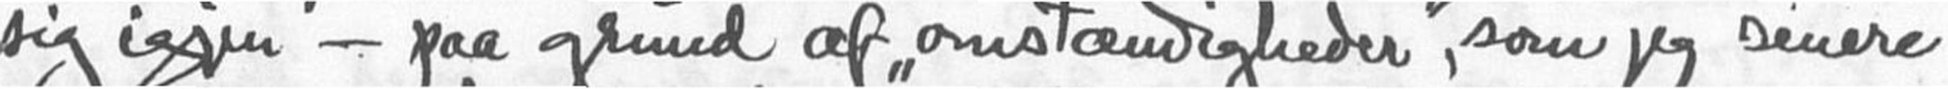sig igjen - paa grund af "omstændigheder", som jeg senere
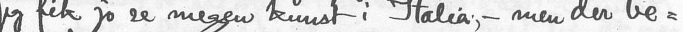Jeg fik jo se megen kunst i Italia, - men der be-
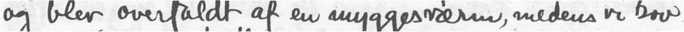og blev overfaldt af en myggesværm, medens vi sov
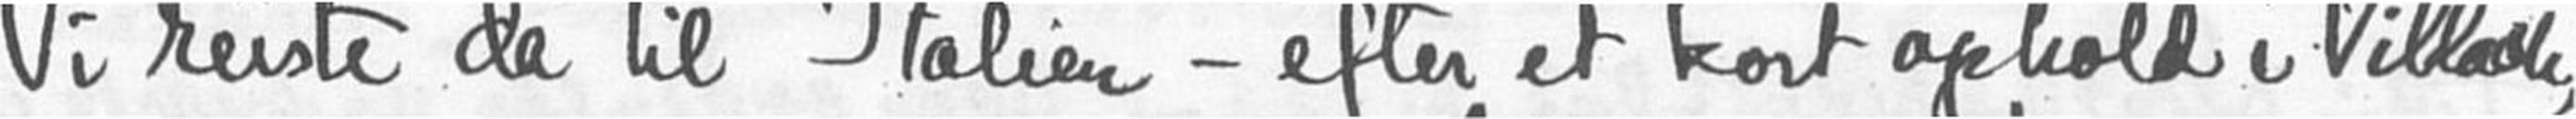Vi reiste da til Italien - efter et kort ophold i Villach,
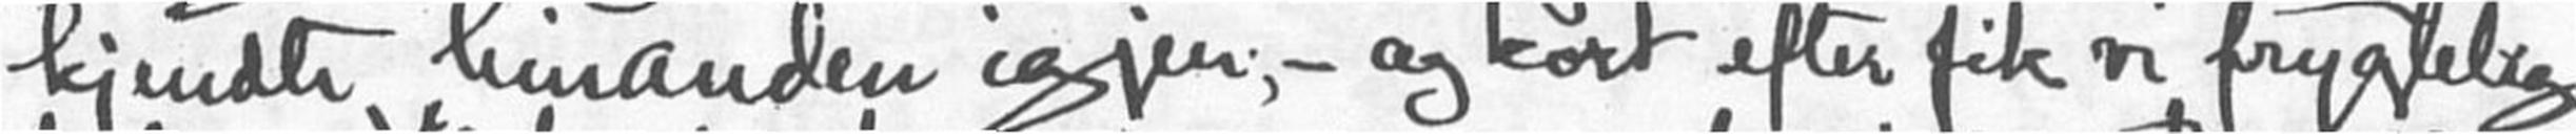kjendte hinanden igjen, - og kort efter fik vi frygtelig
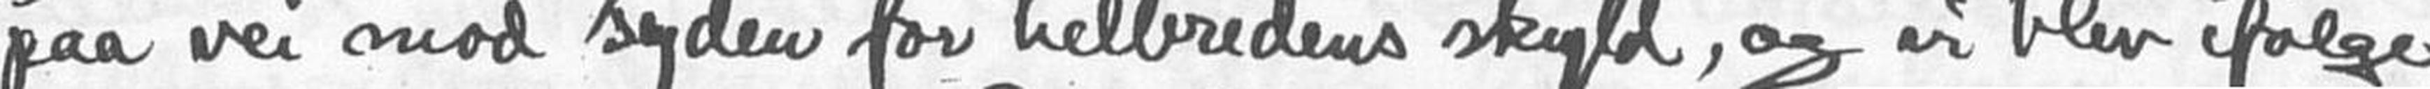paa vei mod syden for helbredens skyld, og vi blev ifølge
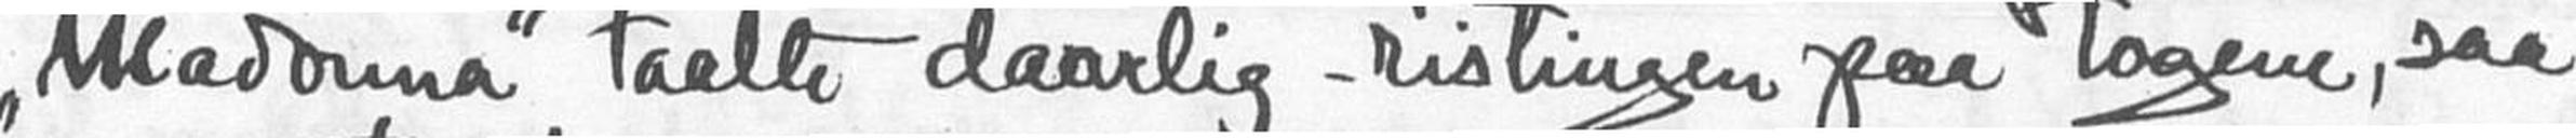"Madonna" taalte daarlig ristingen paa togene, saa
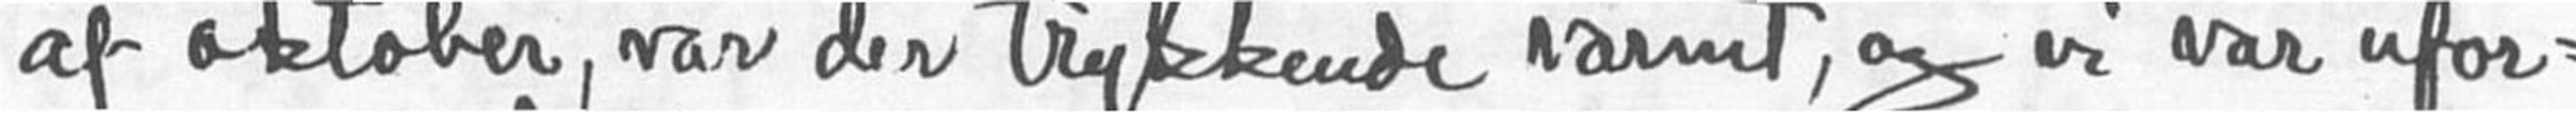af oktober, var der trykkende varmt, og vi var ufor-
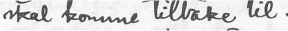skal komme tilbage til. -
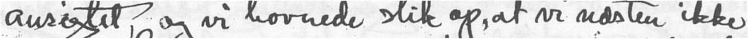ansigtet, og vi hovnede slik op, at vi næsten ikke
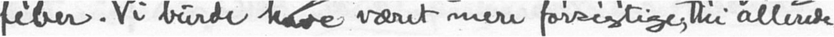feber. Vi burde have været mere forsigtige; thi allerede
